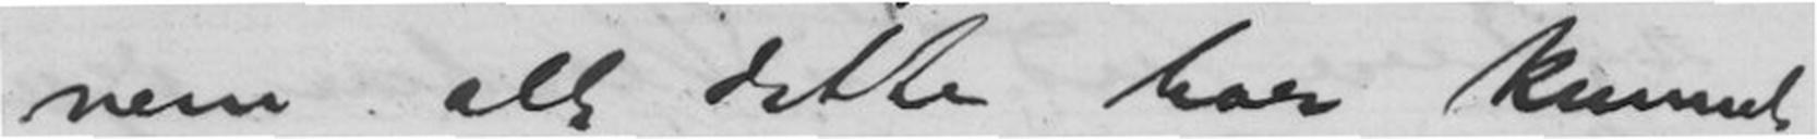nem alt dette har kunnet
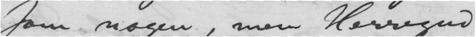som nogen, men Herregud
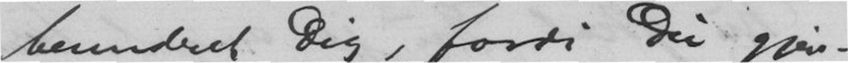beundret Dig, fordi Du gjen-
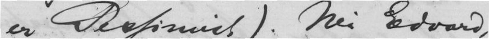er Pessimist). Nei Edvard,
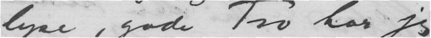lyse, gode Tro har jeg
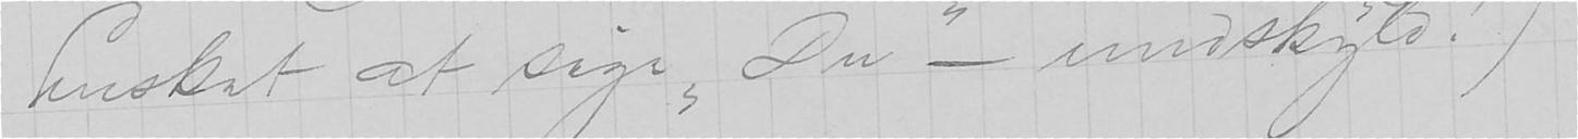husket at sige "Du" - undskyld!)
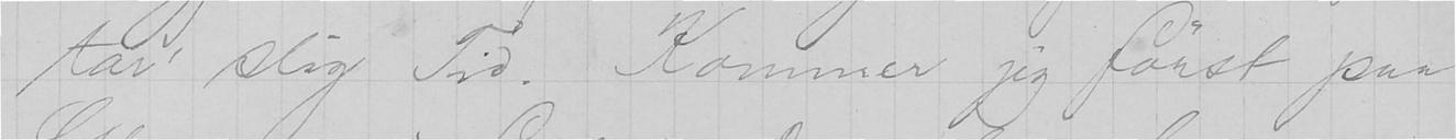tar slig Tid. Kommer jeg først paa
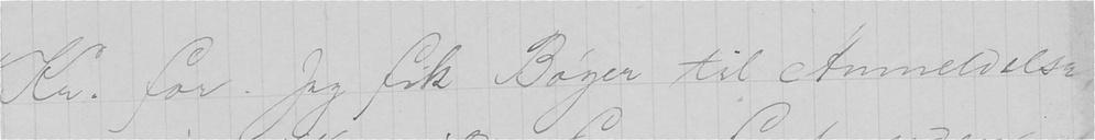Kr. for. Jeg fik Bøger til Anmeldelse
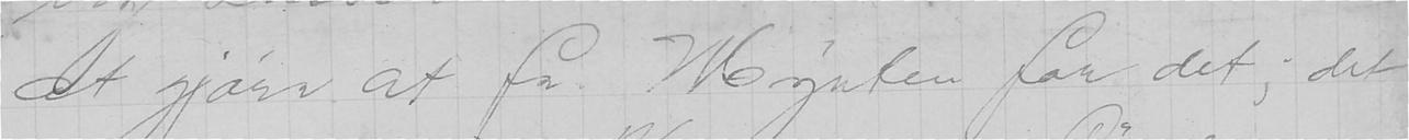at gjøre at faa Mynter for det; det
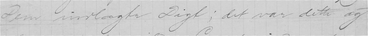Dem indlagte Digt; det var dette og
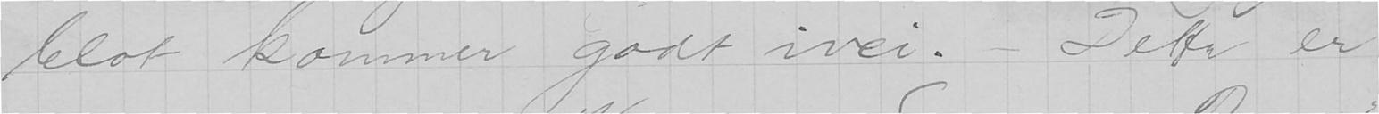blot kommer godt ivei. - Dette er
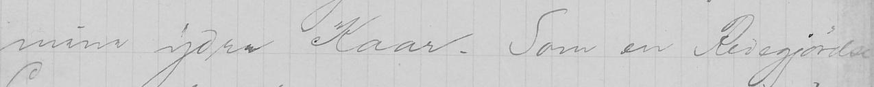mine ydre Kaar. Som en Redegjørelse
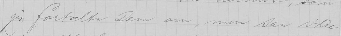jeg fortalte Dem om, men saa vilde
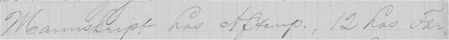Manuskript hos Aftenp., 12 hos Fær-
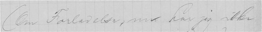(Om Forladelse, nu har jeg ikke
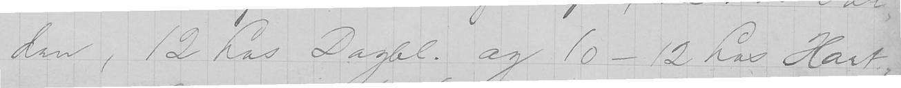den, 12 hos Dagbl. og 10-12 hos Hart-
